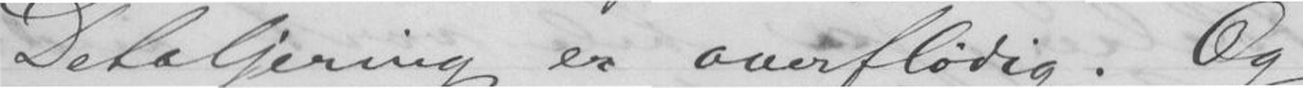Detaljering er overflødig. Og
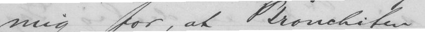mig for, at Bronchiten
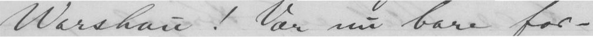Warshau! Vær nu bare for-
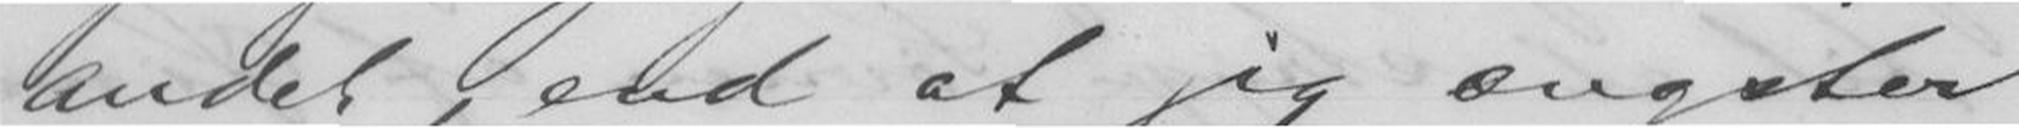andet, end at jeg ængster
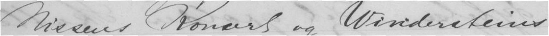Nissens Konsert og Windersteins
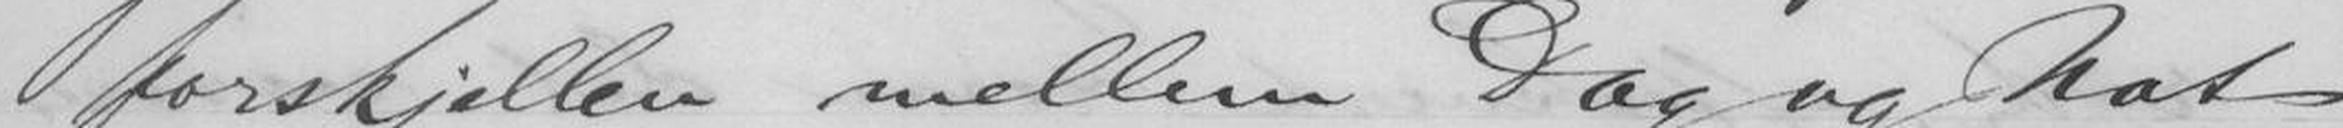forskjellen mellem Dag og Nat
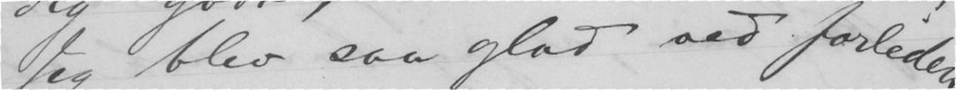Jeg blev saa glad ved forleden
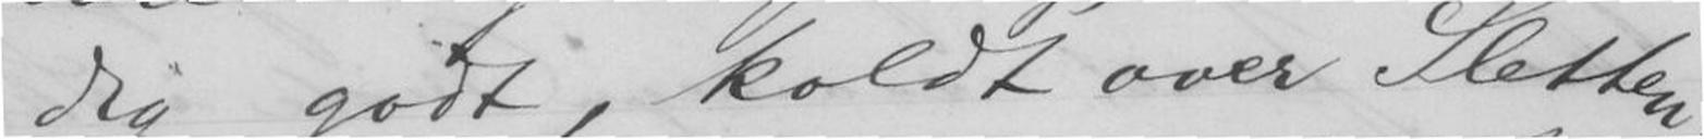Dig godt, koldt over Sletten!"
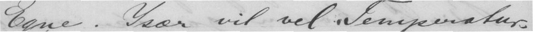Egne. Især vil vel Temperatur-
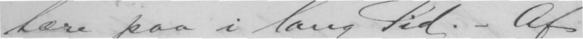tære paa i lang Tid. - Af
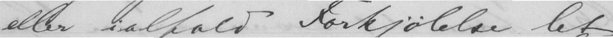eller ialfald Forkjølelse let
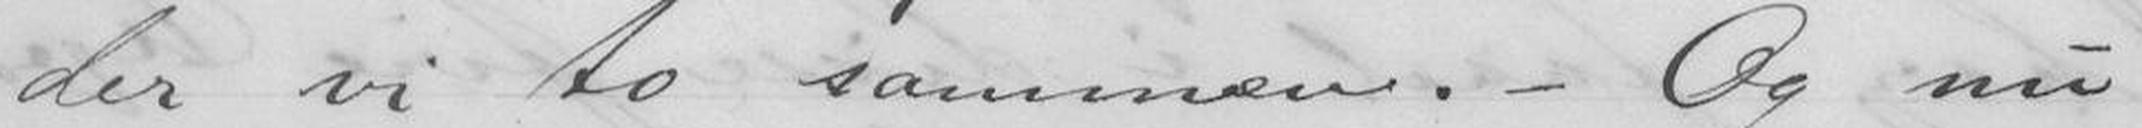der vi to sammen. - Og nu
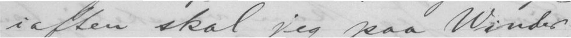iaften skal jeg paa Winder-
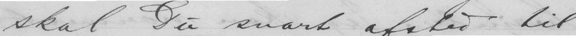skal Du snart afsted til
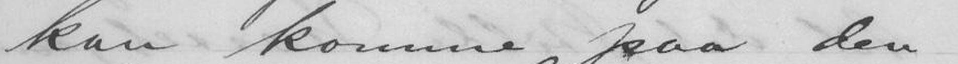kan komme paa den
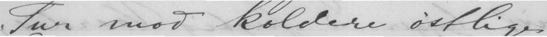Tur mod koldere østlige
